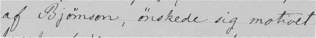af Bjørnson, ønskede sig motivet
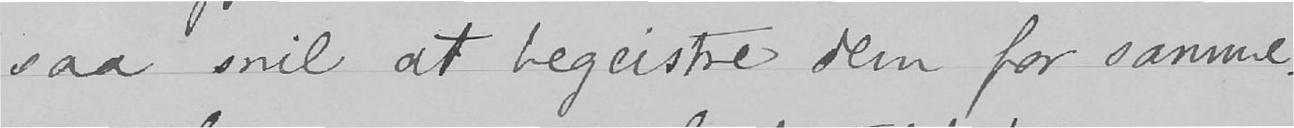saa snil at begeistre dem for samme.
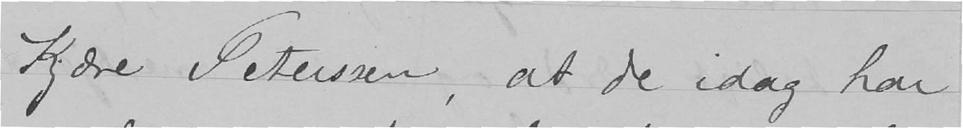Kjære Peterssen, at de idag har
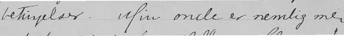betingelser. Min oncle er nemlig me-
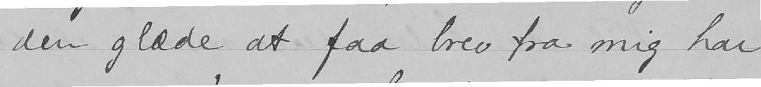den glæde at faa brev fra mig har
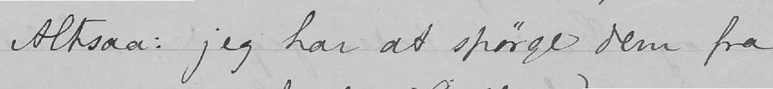Altsaa: jeg har at spørge dem fra
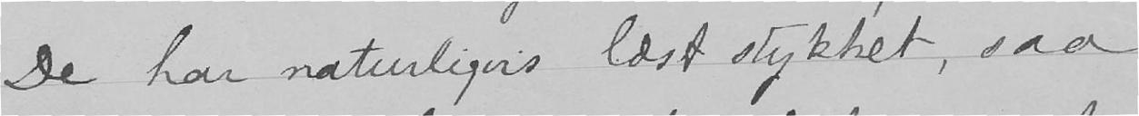De har naturligvis læst stykket, saa
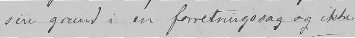sin grund i en forretningssag og ikke
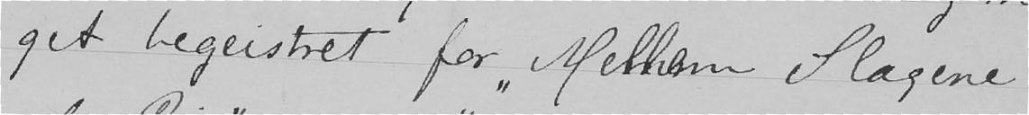get begeistret for «Mellem Slagene»
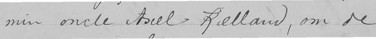min oncle Axel Kielland, om de
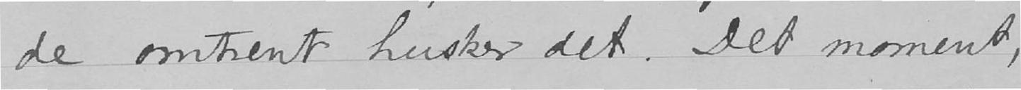de omtrent husker det. Det moment,
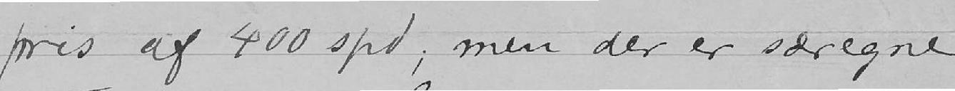pris af 400 spd; men der er særegne
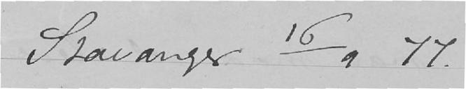Stavanger 16/9 77.
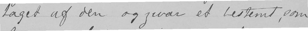taget af den og zwar et bestemt, som
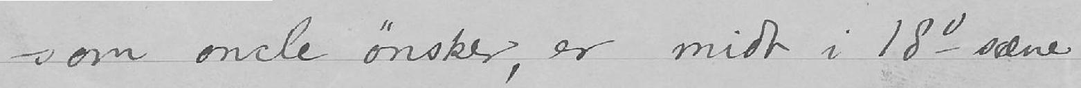som oncle ønsker, er midt i 18d scene
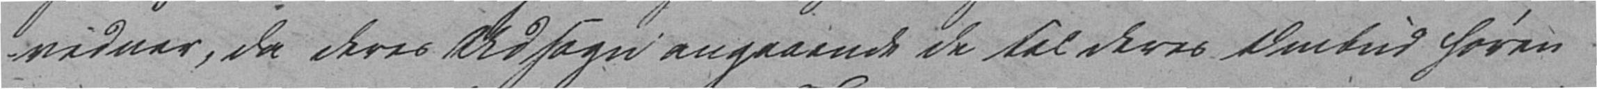vidner, da deres Udsagn angaaende de til deres Ombud høren-
